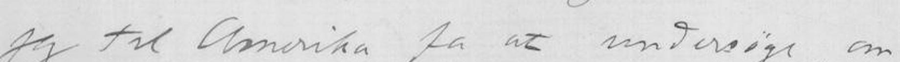jeg til Amerika for at undersøge, om
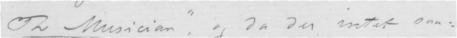"Th Musician", og da der intet saa-
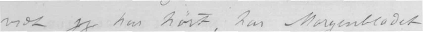vidt jeg har hørt, har Morgenbladet
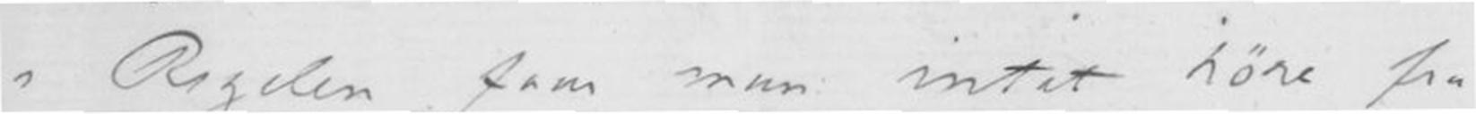i Regelen faar man intet høre fra
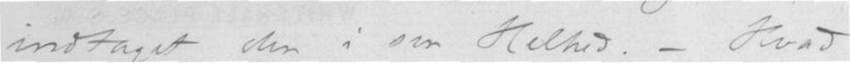indtaget den i sin Helhed. - Hvad
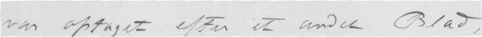var optaget efter et andet Blad,
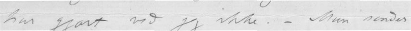har gjort ved jeg ikke. - Man sender
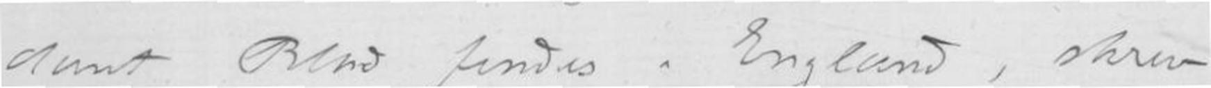dant Blad findes i England, skrev
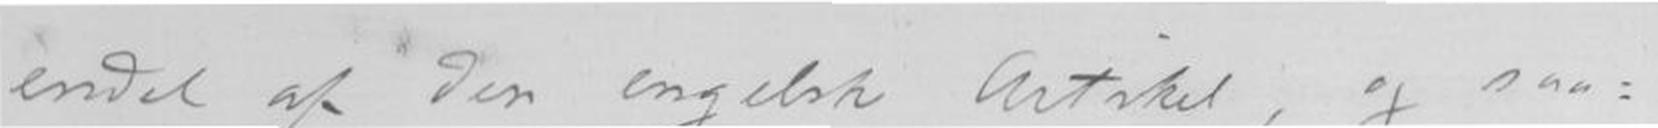endel af Den engelsk Artikel, og saa-
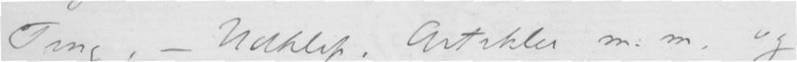Ting, - Udklip. Artikler m.m. og
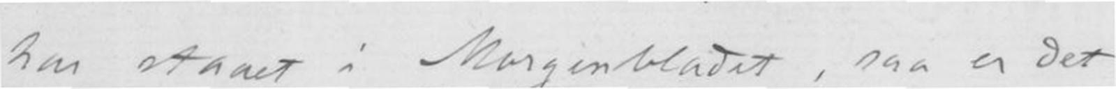har staaet i Morgenbladet, saa er det
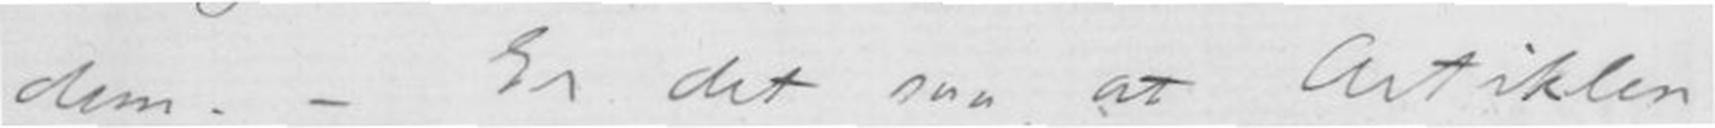dem. - Er det saa, at Artiklen
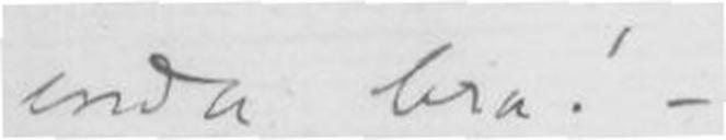enda bra! -
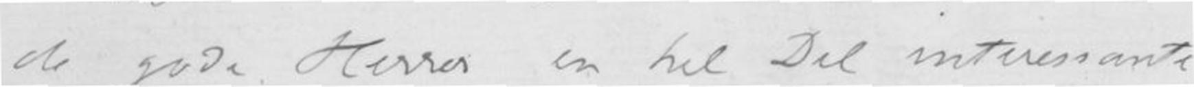de gode Herrer en hel Del interessante
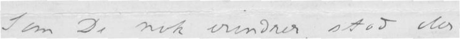Som De nok erindrer, stod der
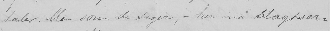taler. Men som de siger, - her må slægtsar-
